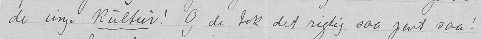de unge kultur! Og de tok det rigtig saa pent saa!
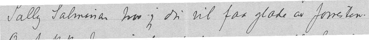Sally Salminen tror jeg du vil faa glæde av forresten.
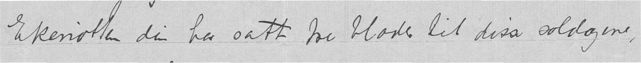Ekenøtten din har satt tre blader til disse soldagene,
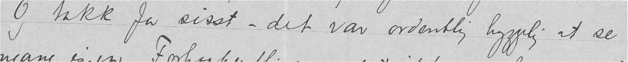Og takk for sisst – det var ordentlig hyggelig at se
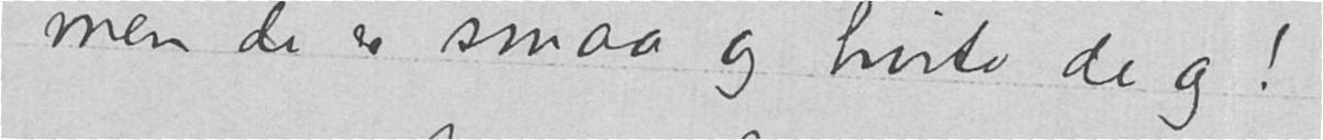- men de er smaa og hvite de og!
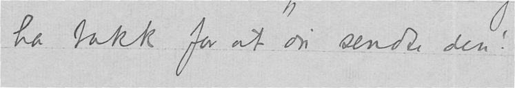ha takk for at du sendte den!
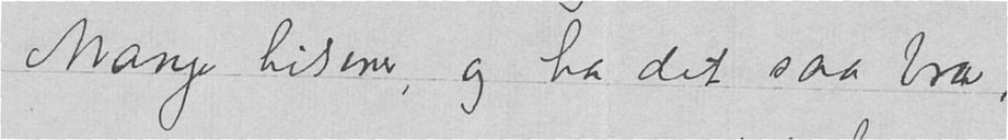Mange hilsener, og ha det saa bra,
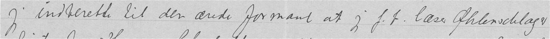jeg indberette til den ærede formand at jeg f.t. læser Øhlenschlæger
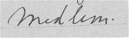medlem.
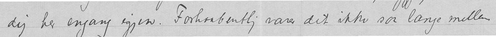dig her engang igjen. Forhaabentlig varer det ikke saa længe mellem
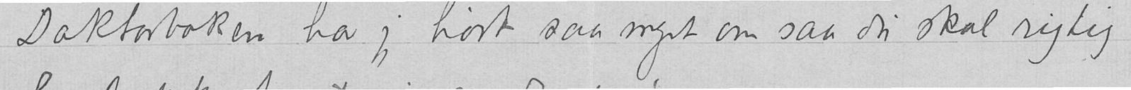Doktorboken har jeg hørt saa meget om saa du skal rigtig
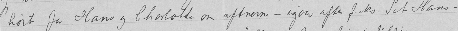høit for Hans og Charlotte om aftnerne – igaar aftes f.eks. Sct Hans-
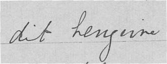dit hengivne
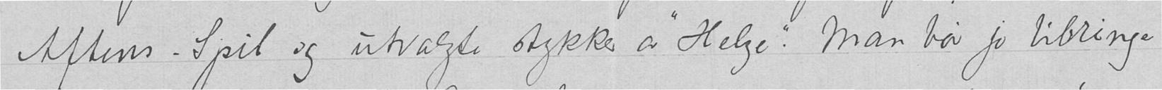Aftens-Spil og utvalgte stykker av "Helge". Man bør jo bibringe
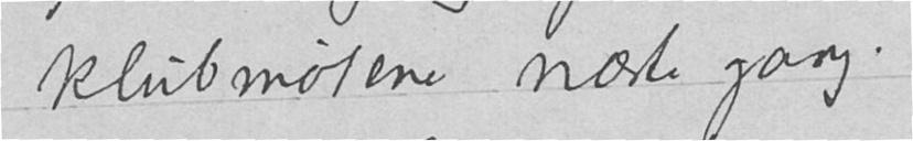klubmøterne næste gang!
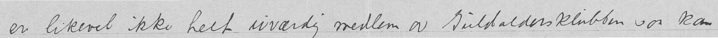er likevel ikke helt uværdig medlem av Guldaldersklubben saa kan
